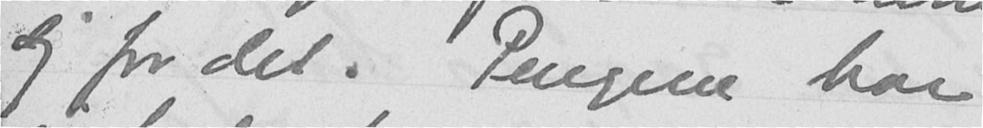sig for det. Pengene har
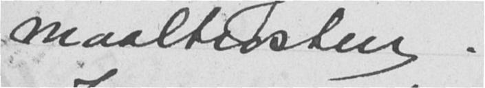maaltrosten.
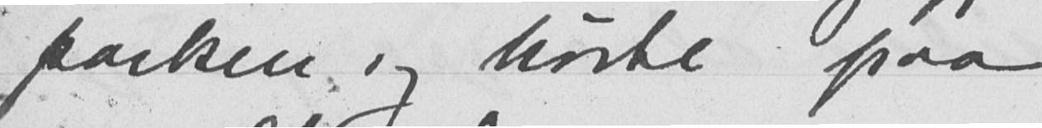parken og hørte paa
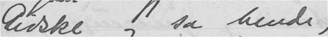Gidske og sa hende,
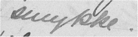smykke.
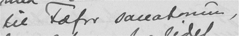til Fæfor sanatorium,
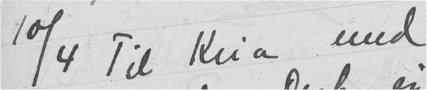10/4 Til Kria med
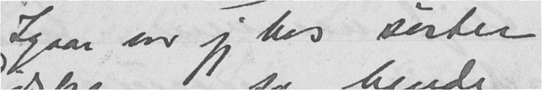Igaar var jeg hos søster
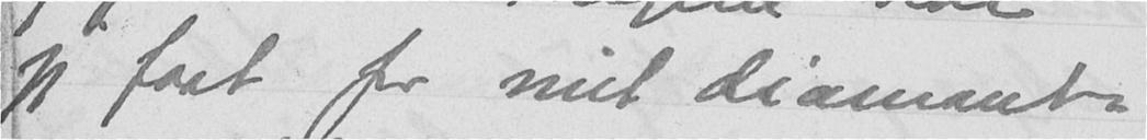jeg faat for mit diamant-
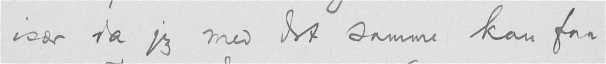især da jeg med det samme kan faa
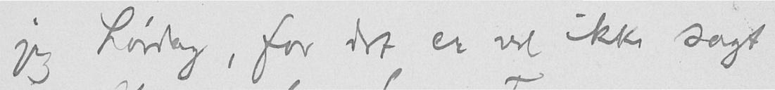jeg Lørdag, for det er vel ikke sagt
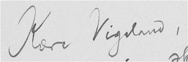Kære Vigeland,
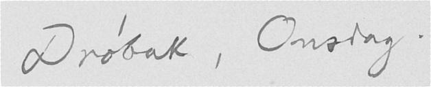Drøbak, Onsdag.
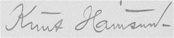Knut Hamsun.
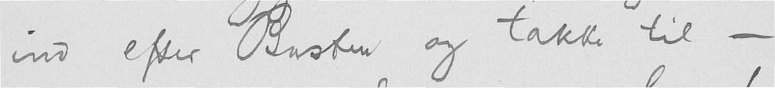ind efter Busten og takke til -
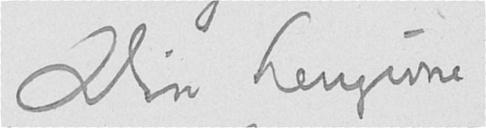Din hengivne
